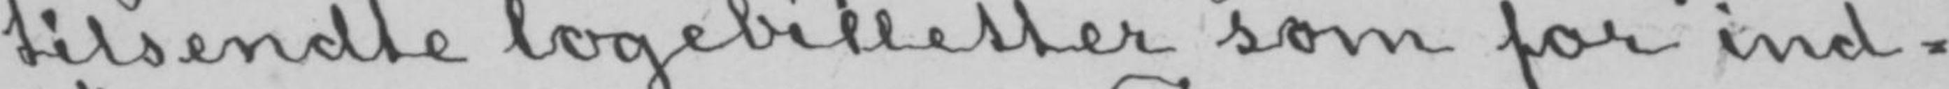tilsendte logebilletter som for ind-
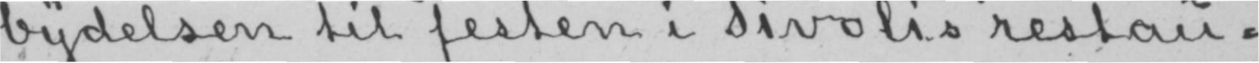bydelsen til festen i Tivolis restau-
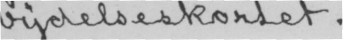bydelseskortet.
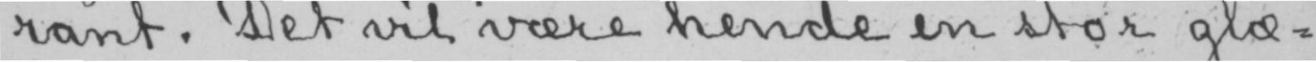rant. Det vil være hende en stor glæ-
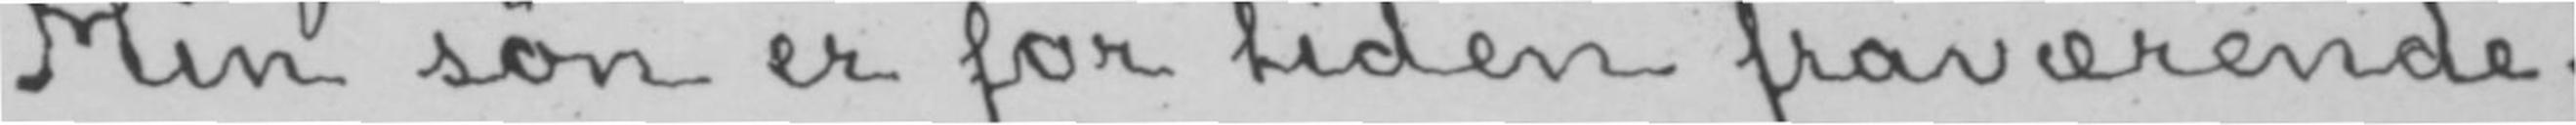Min søn er for tiden fraværende.
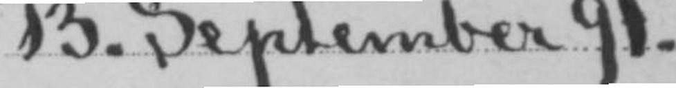13. September 91.
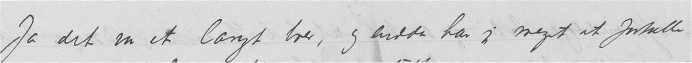Ja det var et langt brev, og endda har jeg meget at fortælle
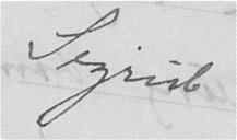Sigrid
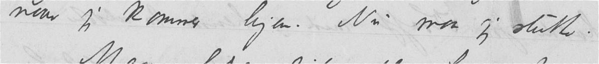naar jeg kommer hjem. Nu maa jeg slutte.
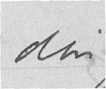din
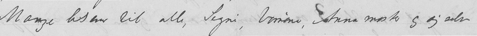Mange hilsener til alle, Signe, børnene, Anna moster og dig selv
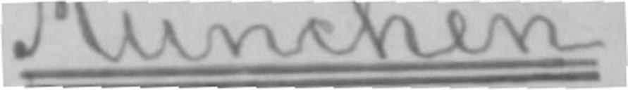München.
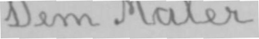Dem Maler
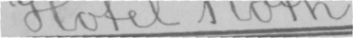Hotel Roth.
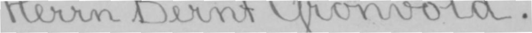Herrn Bernt Grønvold.
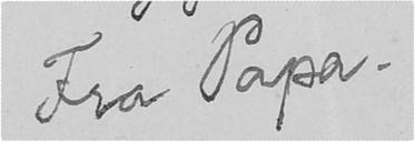Fra Papa.
